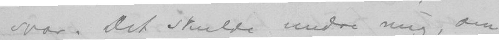svar. Det skulde undre mig, om
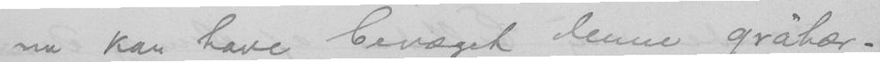nu kan have bevæget denne gråhær-
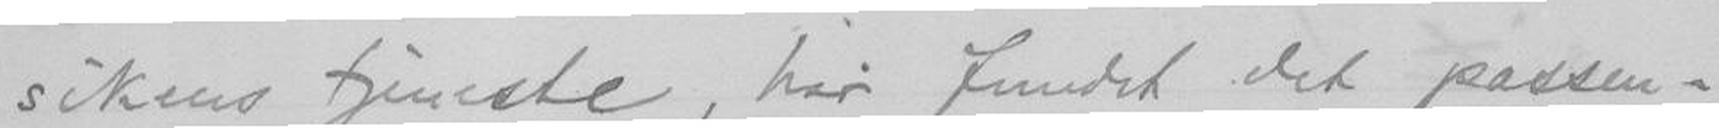sikens tjeneste, har fundet det passen-
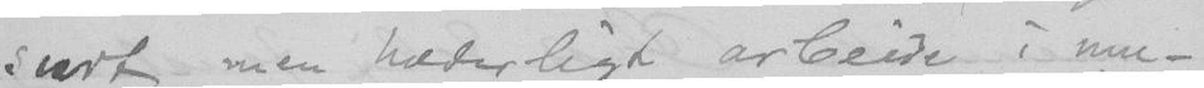svært men hæderligt arbeide i mu-
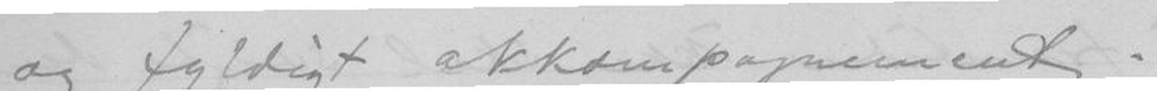og fyldigt akkompagnement.
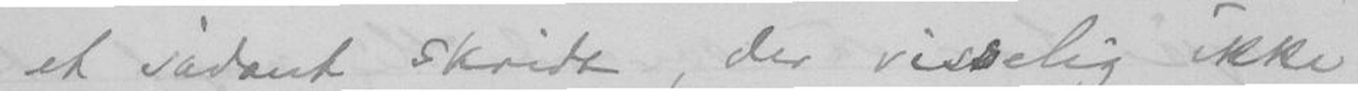et sådant skridt, der visselig ikke
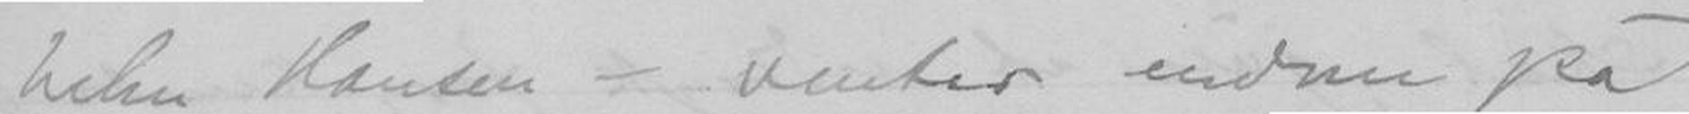helm Hansen - venter endnu på
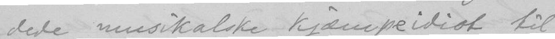dede musikalske kjæmpeidiot til
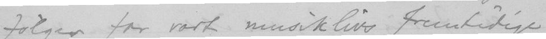følger for vort musiklivs fremtidige
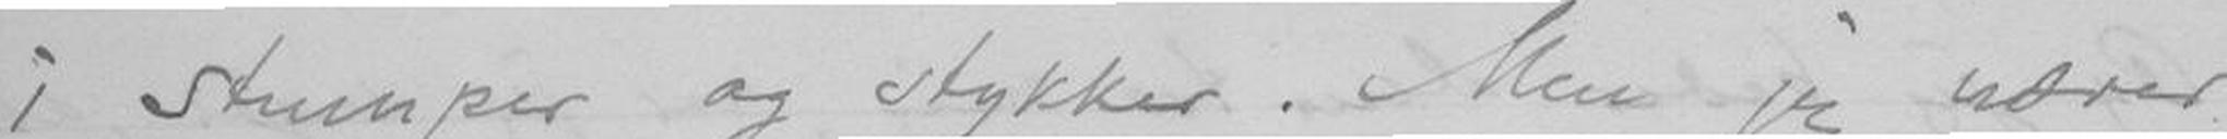i stumper og stykker. Men jeg nærer
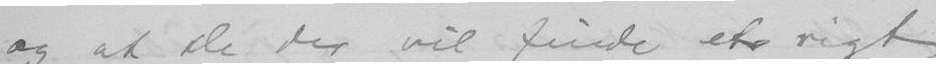og at De der vil finde et rigt
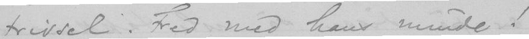trivsel. Fred med hans minde!
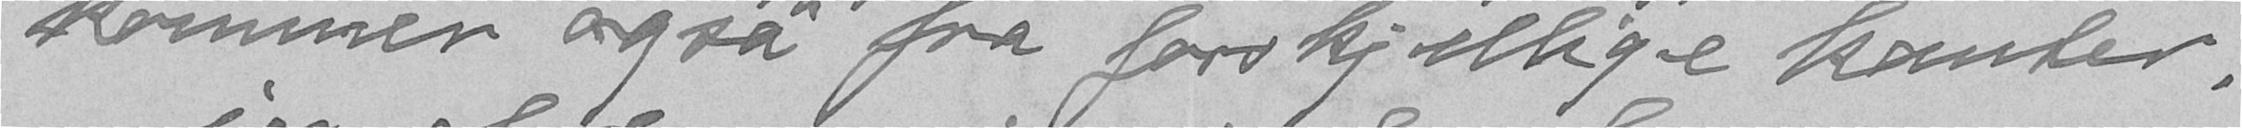kommer også fra forskjellige kanter. -
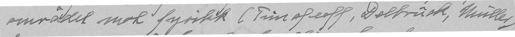området mot fysikk (Timofeeff, Delbrück, Müller)
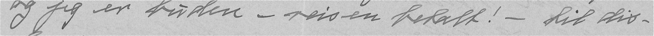og jeg er buden - reisen betalt! - til dis-
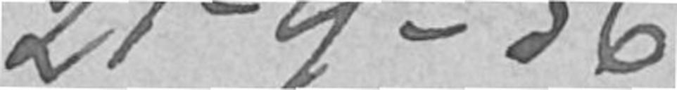21-9-36
